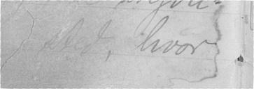sted, hvor
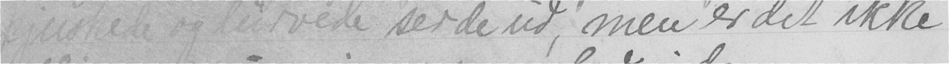pjuskede og lurvede ser de ud, men er det ikke
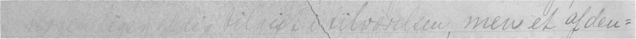tilgift i tilværelsen, men et af den-
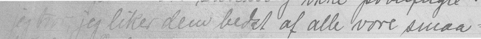jeg tror, jeg liker dem bedst af alle vore smaa-
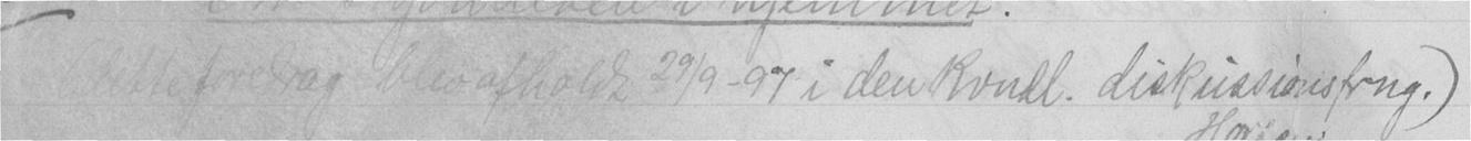(dette foredrag blev afholdt 29/9-97 i den kvndl. diskussionsfrng.)
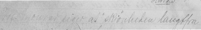Prod. Monrad siger, at "skjønheden langtfra
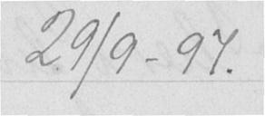29/9-97.
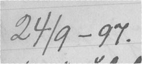24/9-97.
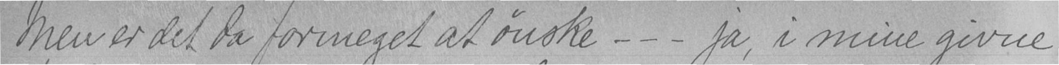Men er det da formeget at ønske - - - ja, i mine givne
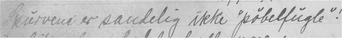Spurvene er sandelig ikke "pøbelfugle"!
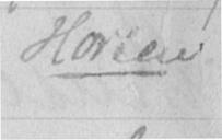Horten
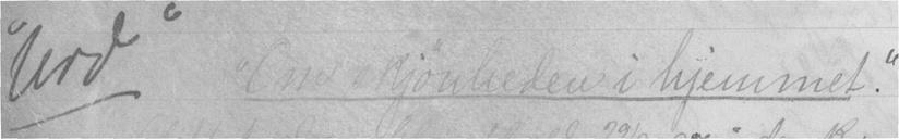"Urd"- "Om skjønheden i hjemmet."
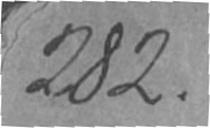282.
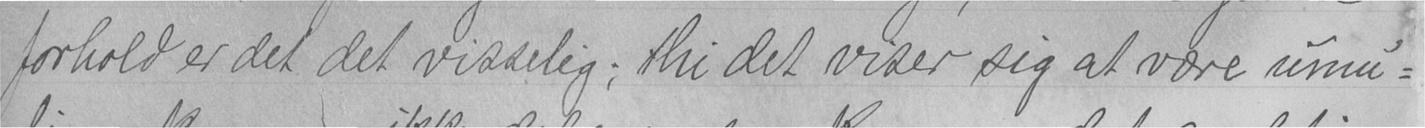forhold er det det visselig; thi det viser sig at være umu-
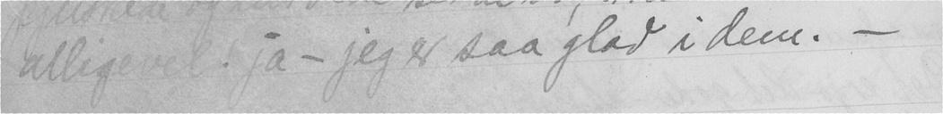alligeve! ja - jeg er saa glad i dem. -
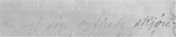vi vil søge of finde og skjøn-
